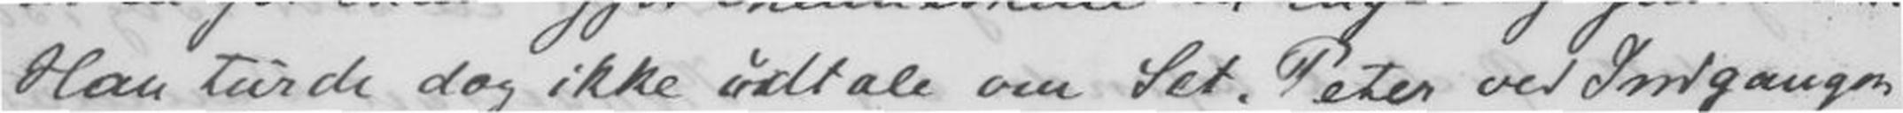Han turde dog ikke udtale om Sct. Peter ved Indgangen
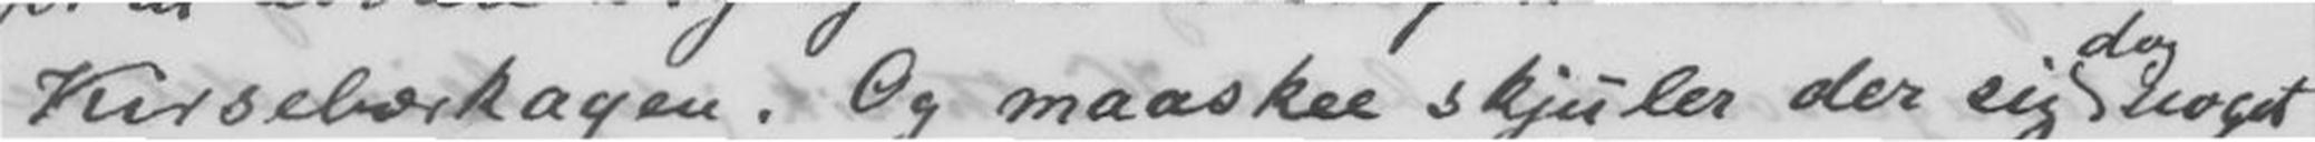Kirsebærkagen. Og maaskee skjuler der sig Noget
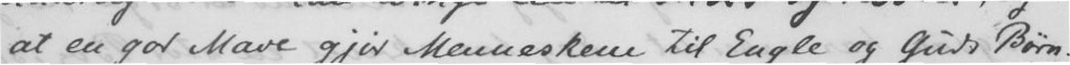at en god Mave gjør Menneskene til Engle og Guds Børn.
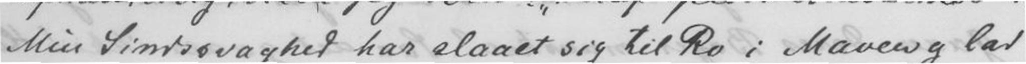Min Sindssvaghed har slaaet sig til Ro i Maven og lad
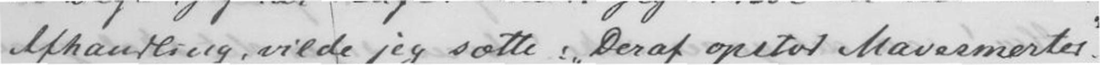Afhandling vilde jeg sætte: Deraf opstod Mavesmerter.
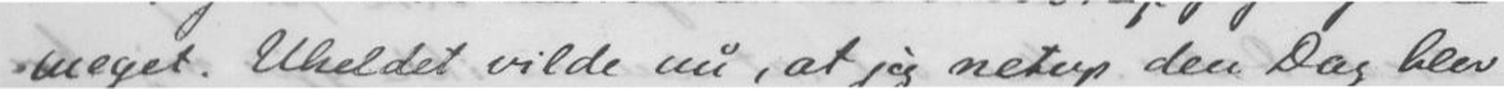meget. Uheldet vilde nu, at jeg netop den Dag blev
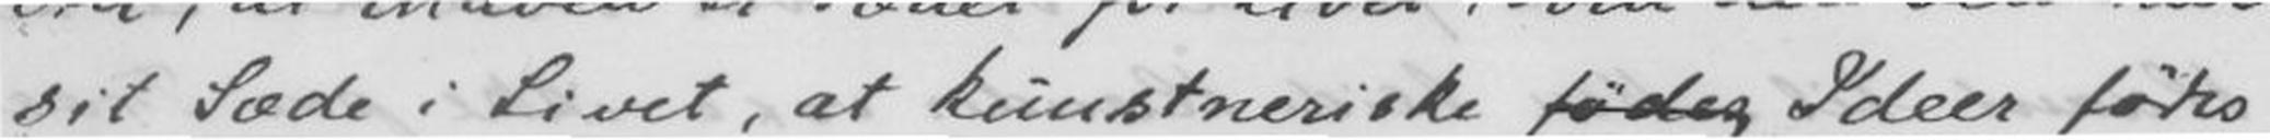sit Sæde i Livet, at kunstneriske fø dig Ideer fødes
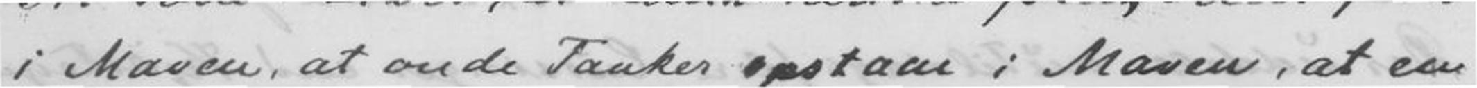i Maven, at onde Tanker opstaae i Maven, at en
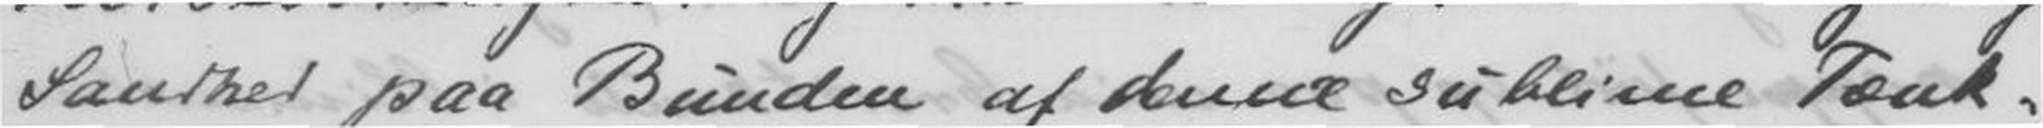Sandhed paa Bunden af denne sublime Tænk-
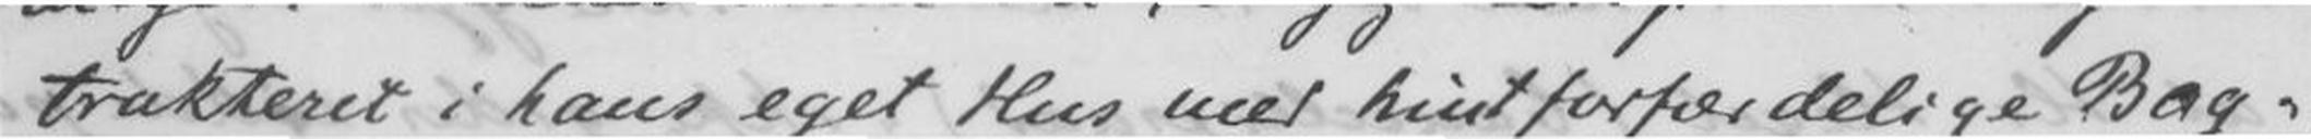trakteret i hans eget Hus med hintforfærdelige Bog-
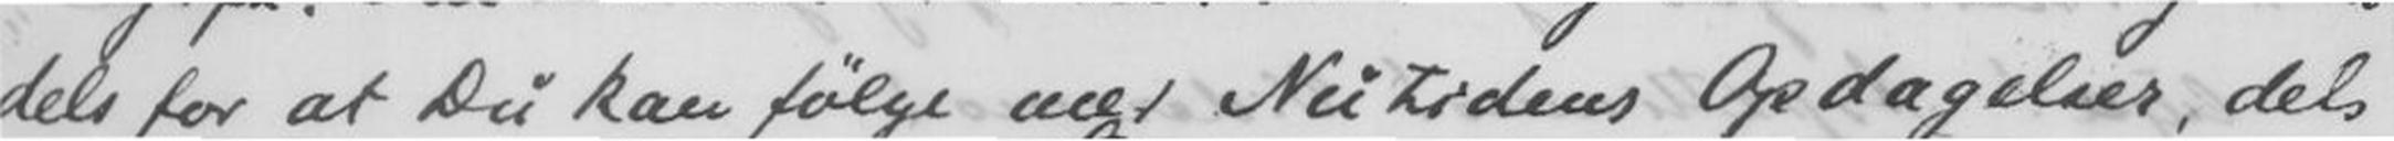dels for at Du kan følge med Nutidens Opdagelser, dels
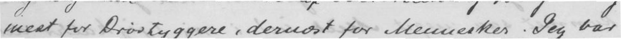mest for Drøvtyggere, dernæst for Mennesker. Jeg var
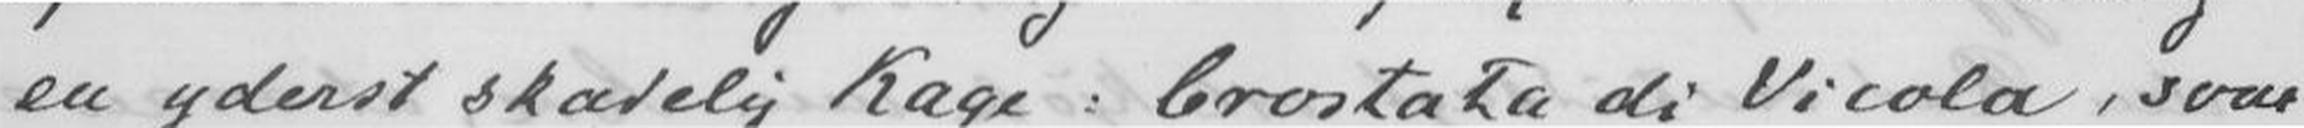en yders skadelig Kage: Crostata di Vicola, svar
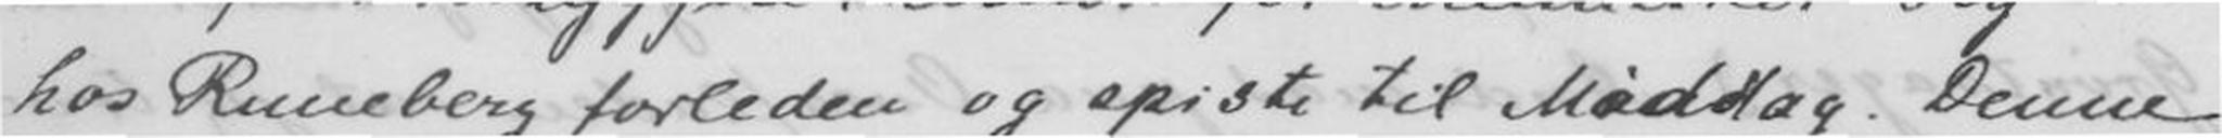hos Runeberg forleden og spiste til Middag. Denne
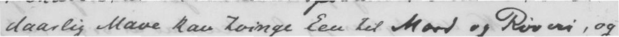daarlig Mave kan tvinge Een til Mord og Røveri, og
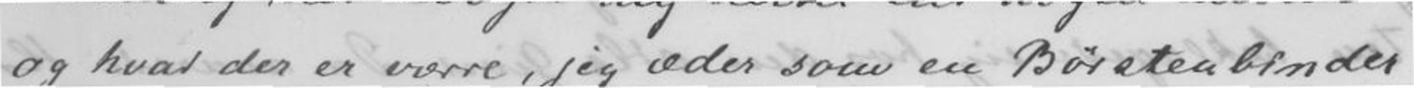og hvad der er værre, jeg æder som en Børstenbinder
